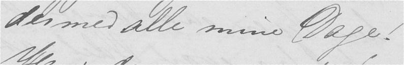dermed alle mine Dage!
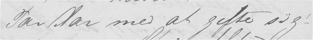Par Aar med at gifte sig.
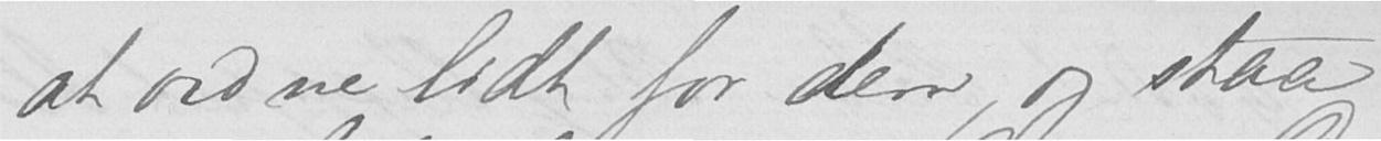at ordne lidt for dem, og staa
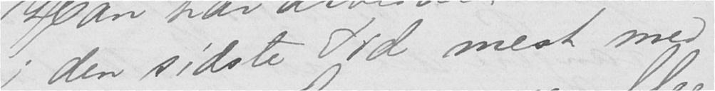i den sidste Tid mest med
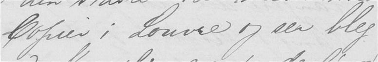Copier i Louvre og ser bleg
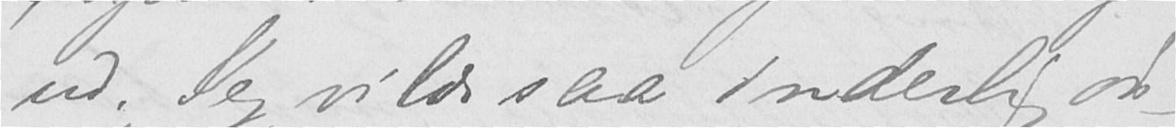ud. Jeg vilde saa inderlig øn-
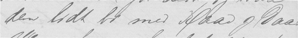dem lidt bi med Raad og Daad.
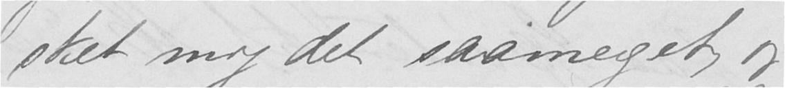sket mig det saameget, og
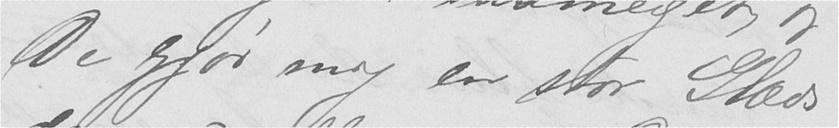De gjør mig en stor Glæde
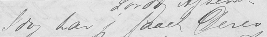Idag har jeg faaet Deres
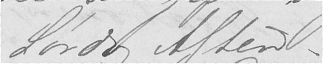Lørdag Aften.
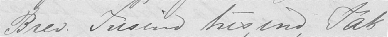Brev. Tusind tusind Tak
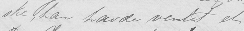ske, han havde ventet et
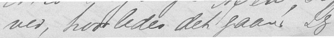ved, hvorledes det gaar! Jeg
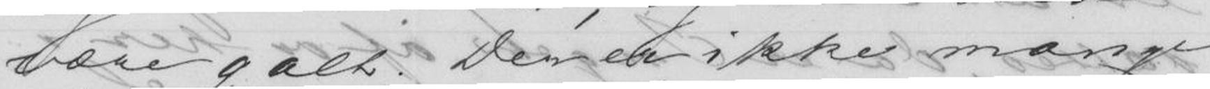være galt. Der ikke mange
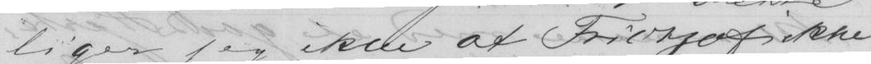liger jeg ikke at Fridtjof ikke
